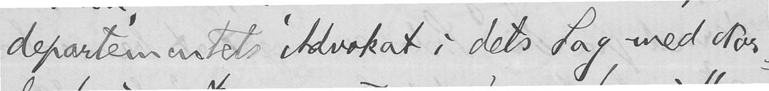departementets Advokat i dets Sag med Nor-
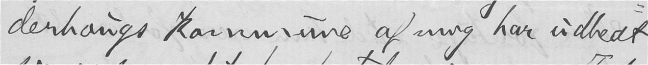derhaugs kommune af mig har udbedt
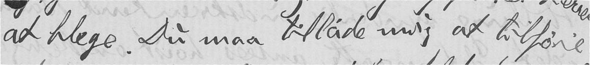at blege. Du maa tillade mig at tilføie,
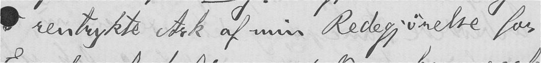rentrykte Ark af min Redegjørelse for
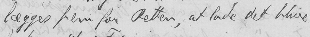lægges frem for Retten, at lade det blive
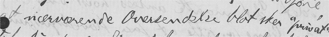at nærværende Oversendelse blot sker "privat"
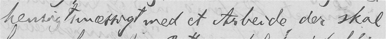hensigtsmæssigt med et Arbeide, der skal
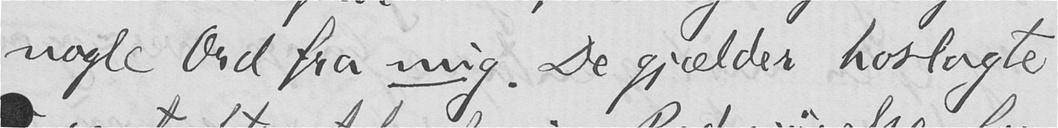nogle Ord fra mig. De gjælder hoslagte
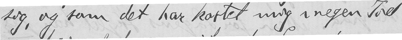sig, og som det har kostet mig megen Tid
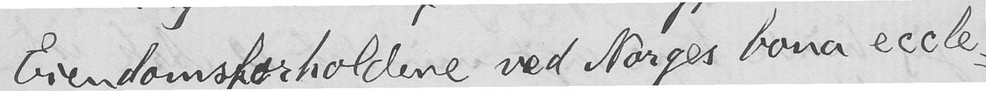eiendomsforholdene ved Norges bona eccle-
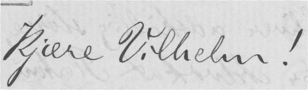Kjære Vilhelm!
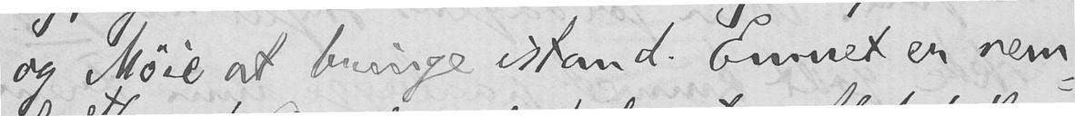og Møie at bringe istand. Emnet er nem-
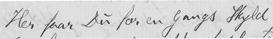Her faar Du for en Gangs Skyld
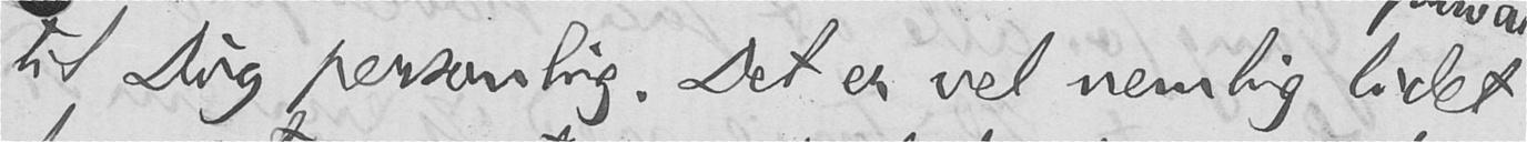til Dig personlig. Det er vel nemlig lidet
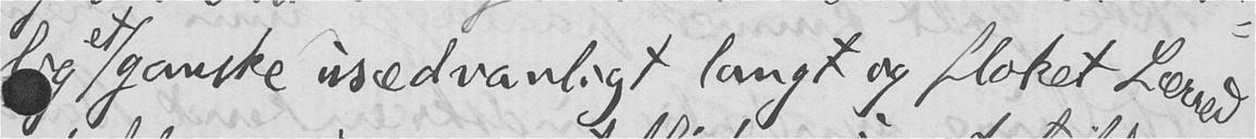lig et ganske usædvanligt langt og floket Lærred
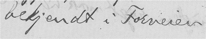bekjendt i Forveien.
